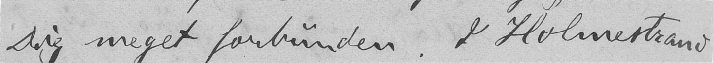Dig meget forbunden. I Holmestrand
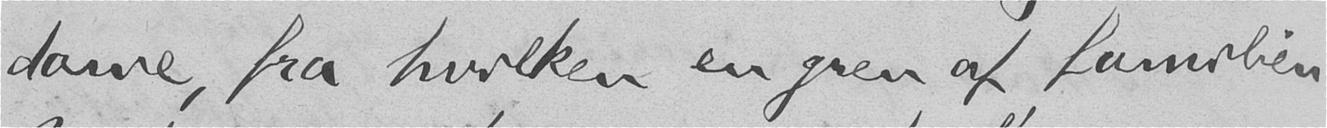dame, fra hvilken en gren af familien
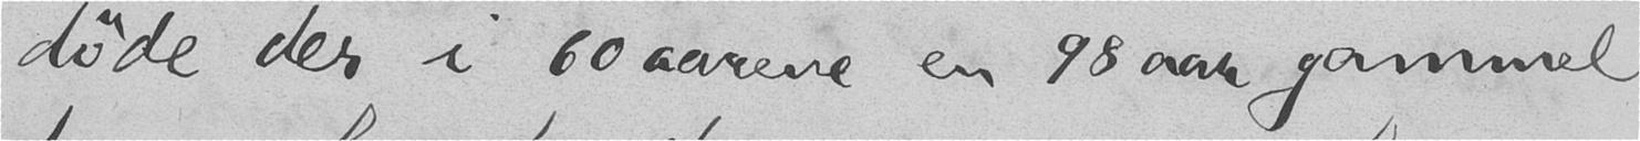døde der i 60 aarene en 98 aar gammel
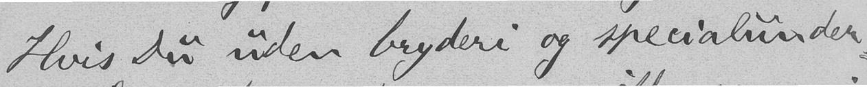Hvis Du uden bryderi og specialunder-
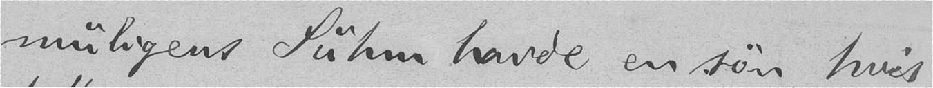muligens Suhm havde en søn, hvis
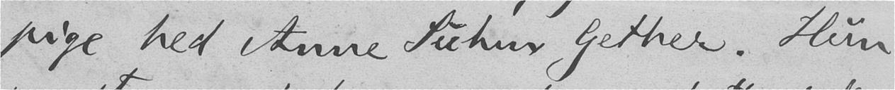pige hed Anne Suhm Gether. Hun
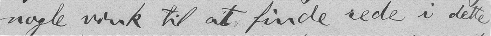nogle vink til at finde rede i dette
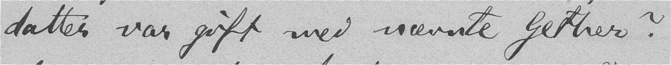datter var gift med nævnte Gether?
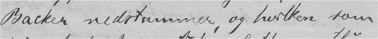Backer nedstammer, og hvilken som
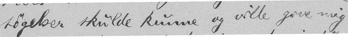søgelser skulde kunne og ville give mig
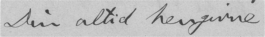Din altid hengivne
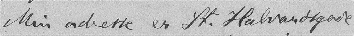Min adresse er St. Halvardsgade
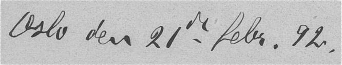Oslo den 21de febr. 92.
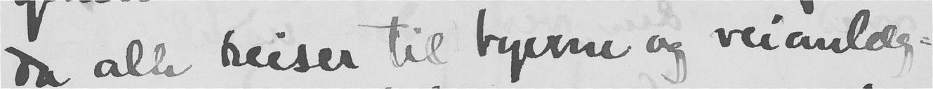da alle reiser til byerne og veianlæg-
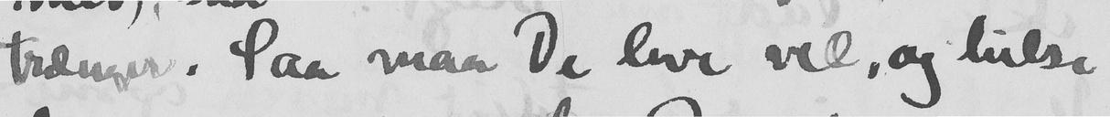trænger. Saa maa De leve vel, og hilse
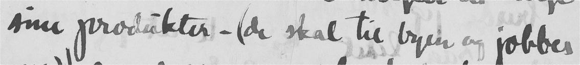sine produkter - (de skal til byen og jobbes
